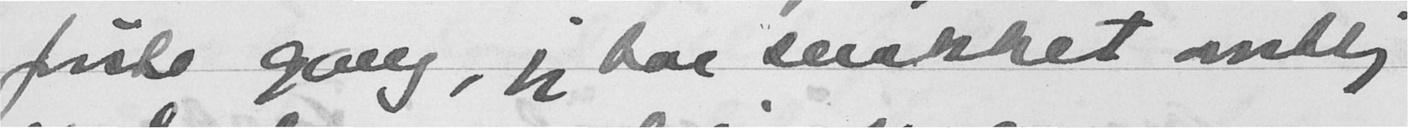første gang, jeg har snakket orntlig
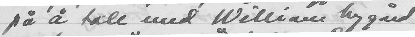på å tale med William Nygård
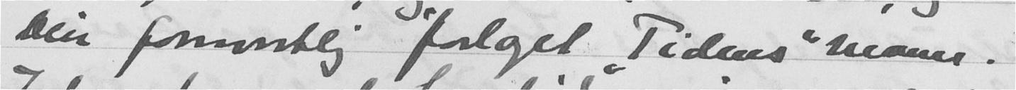blir formentlig forlaget "Tidens" mann.
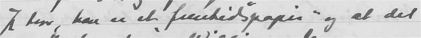Jeg tror han er et fremtidspapir og at det
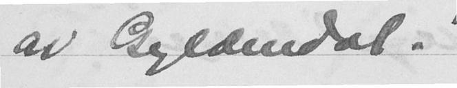av Gyldendal!
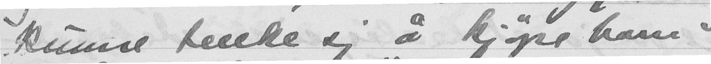kunne tenke sig "å kjøpe ham"
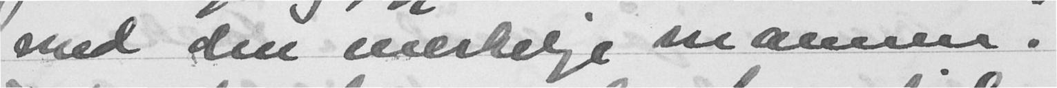med den merkelige mannen.
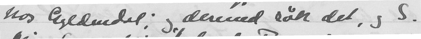hos Gyldendal; og dermed røk det, og S.
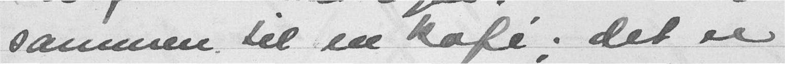sammen til en kafe; det er
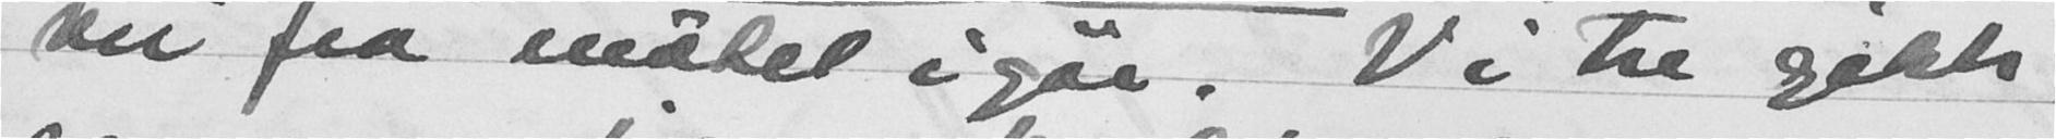vei fra møtet igår. - Vi tre gikk
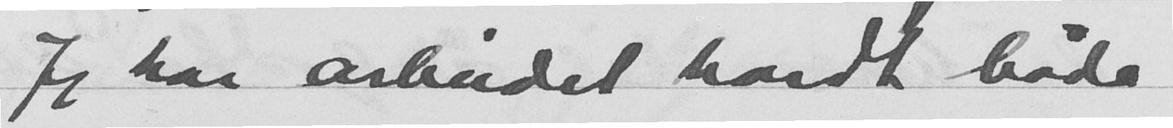Jeg har arbeidet hardt både
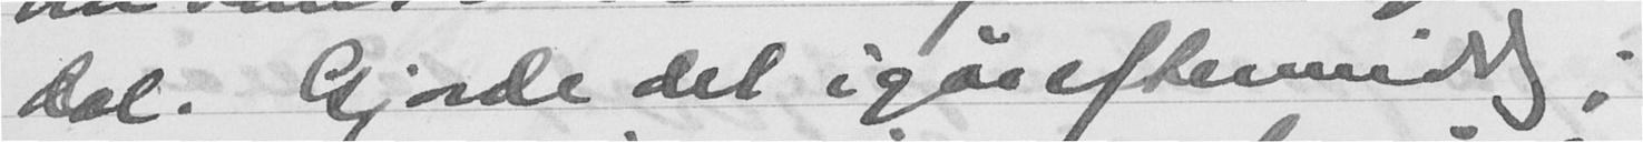dal. Gjorde det igåreftermiddag:
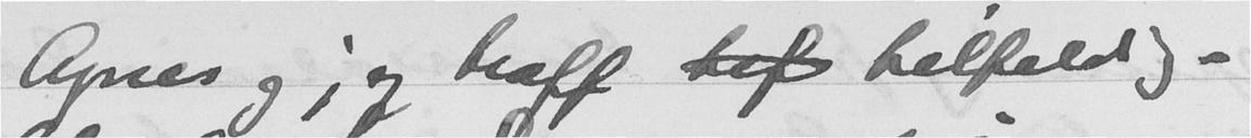Agnes og jeg jeg traff  tilfeldig-
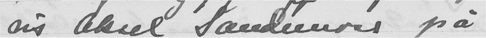vis Aksel Sandemose på
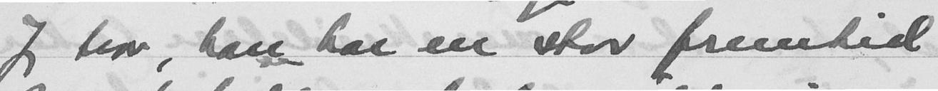Jeg tror, han har en stor fremtid
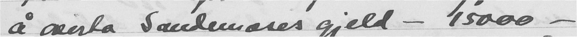å overta Sandemoses gjeld - 15000 -
